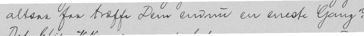altsaa faa træffe Dem endnu en eneste Gang?
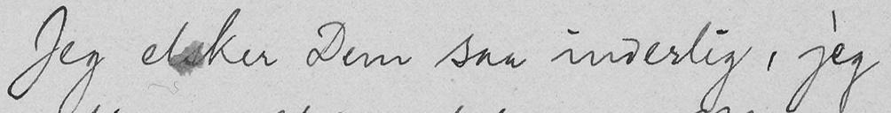Jeg elsker Dem saa inderlig, jeg
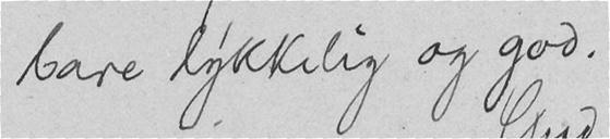bare lykkelig og god.
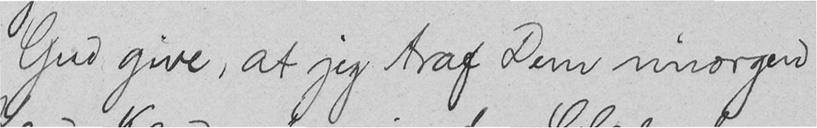Gud give, at jeg traf Dem imorgen
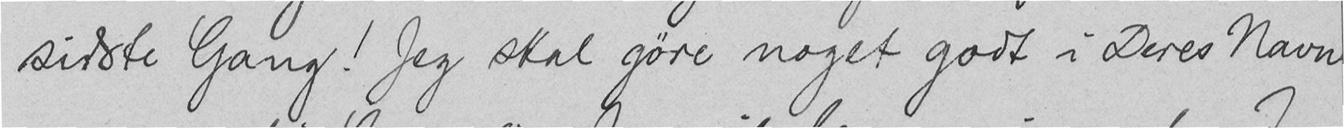sidste Gang! Jeg skal gøre noget godt i Deres Navn
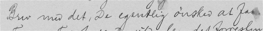Brev med det, De egentlig ønsked at faa.
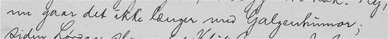nu gaar det ikke længer med Galgenhumor;
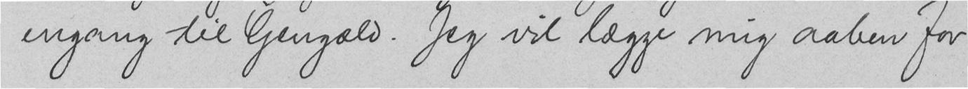engang til Gengæld. Jeg vil lægge mig aaben for
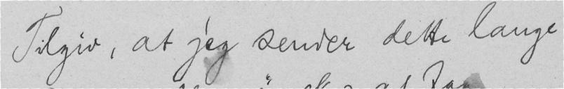Tilgiv, at jeg sender dette lange
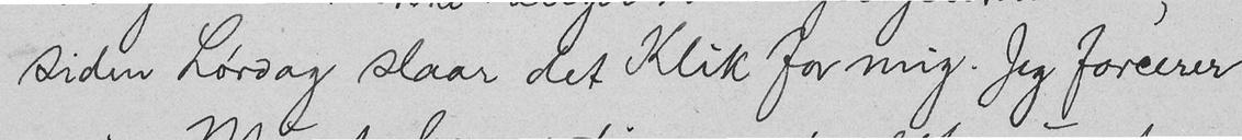siden Lørdag slaar det Klik for mig. Jeg forærer
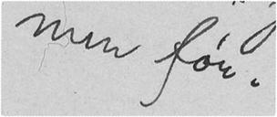men før.
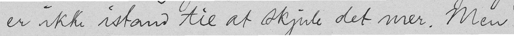er ikke istand til at skjule det mer. Men
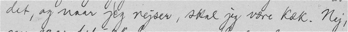det, og naar jeg rejser, skal jeg være kæk. Nej,
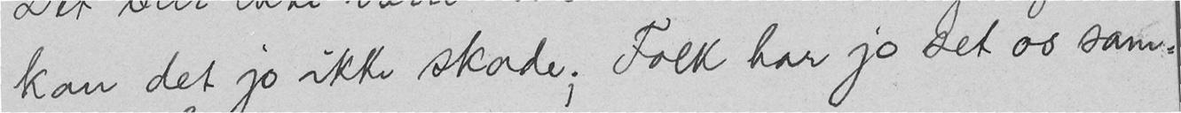kan det jo ikke skade; Folk har jo set os sam-
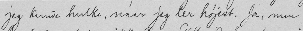jeg kunde hulke, naar jeg ler højest. Ja, men
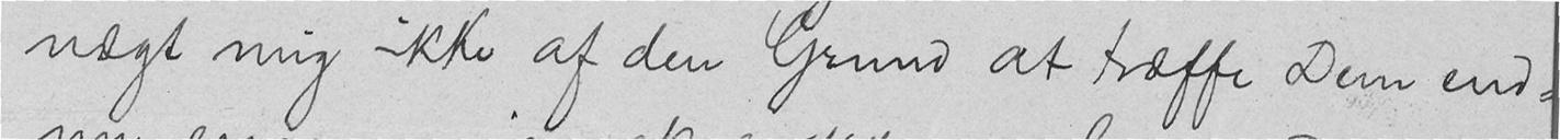nægt mig ikke af den Grund at træffe Dem end-
